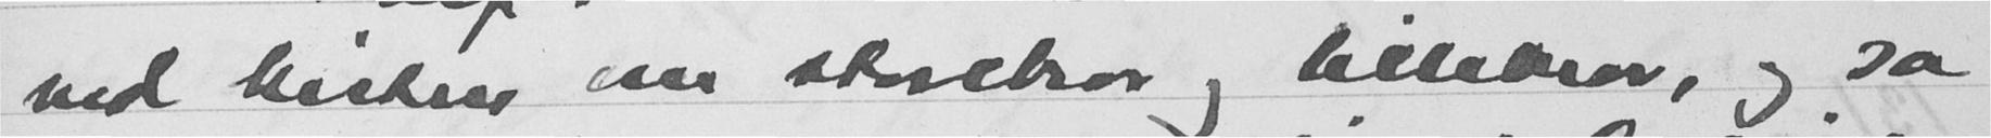ved kisten om storebror og lillebror, og sa
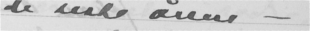de siste årene -
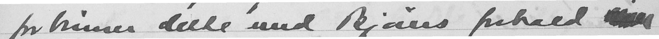forbinner dette med Bjørns forhold
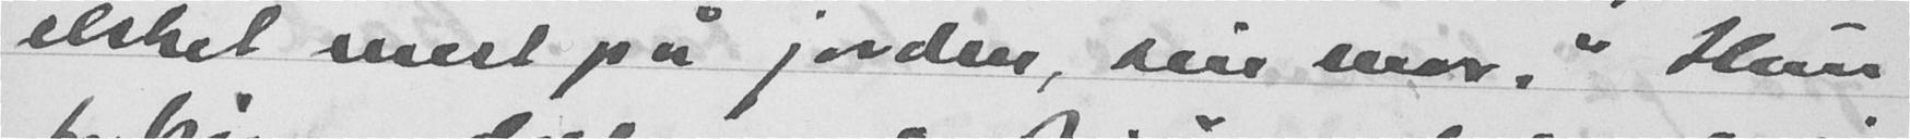elsket mest på jorden, sin mor. "Hun
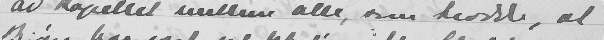av kapellet mellem alle, som trodde, at
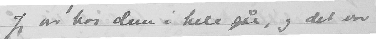Jeg var hos dem i hele går, og det var
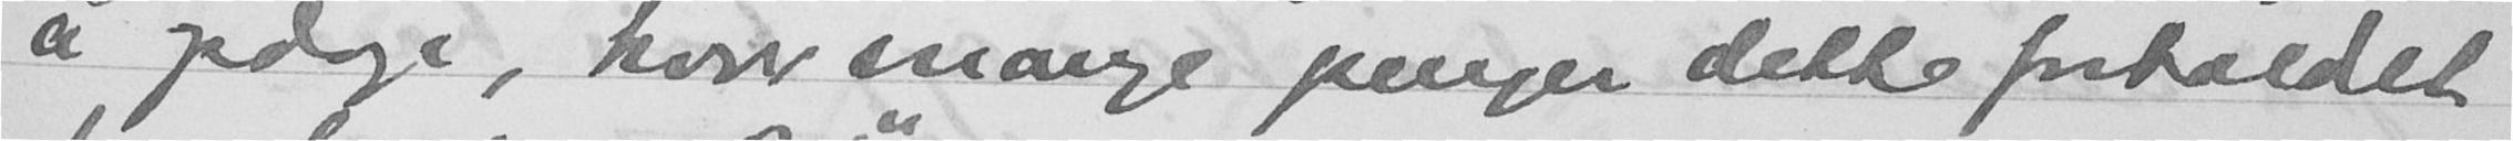å opdage, hvor mange penger dette forholdet
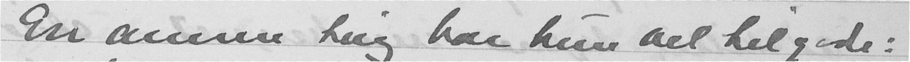En annen ting har hun det til gode:
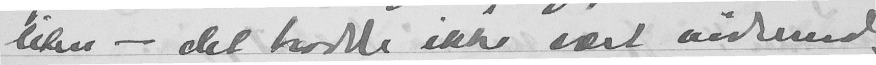liten - det hadde ikke vært anderledes
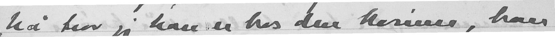Nå tror jeg han er hos den kusinen, han
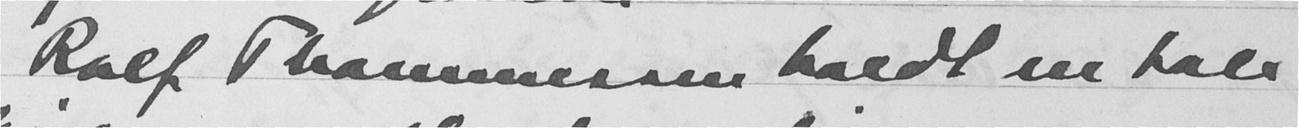Rolf Thommessen holdt en tale
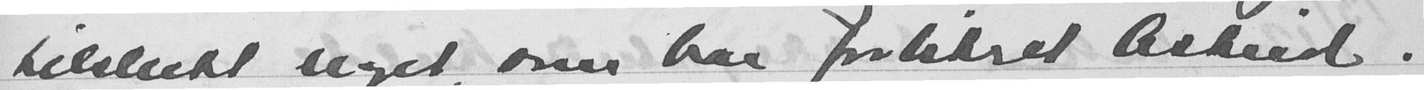tilsludt noget, som har forbitret Asheid.
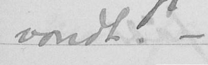vondt. -
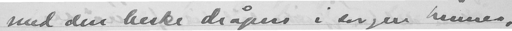med den beske dråpen i sorgen hennes,
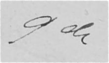9de
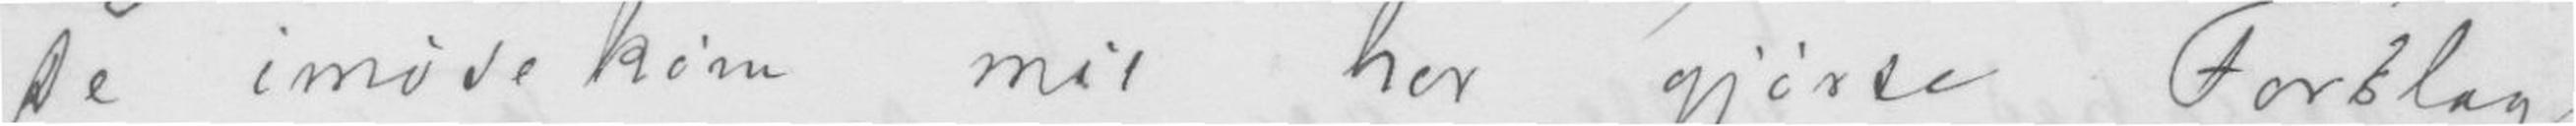De imødekom nu her gjør.. Forslag
